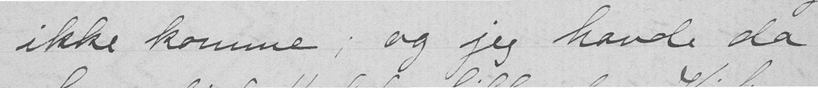ikke komme; og jeg havde da
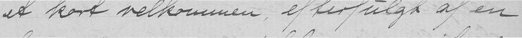et kort velkommen, efterfulgt af en
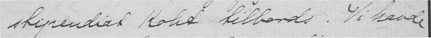stipendiat Koht tilbords. Vi havde
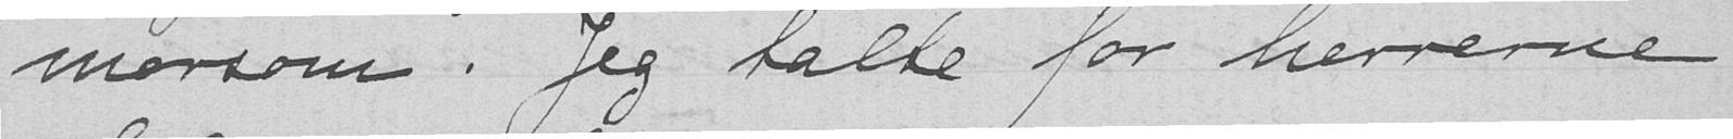morsom. Jeg talte for herrerne
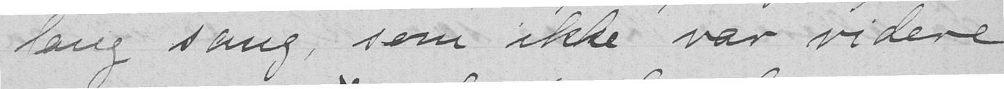lang sang, som ikke var videre
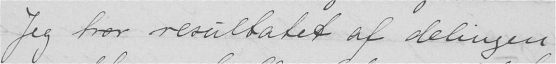Jeg tror resultatet af delingen
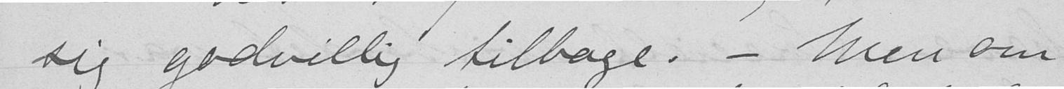sig godvillig tilbage. - Men om
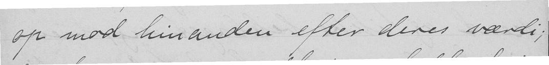op mod hinanden efter deres værdi;
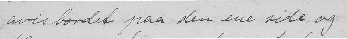avisbordet paa den ene side, og
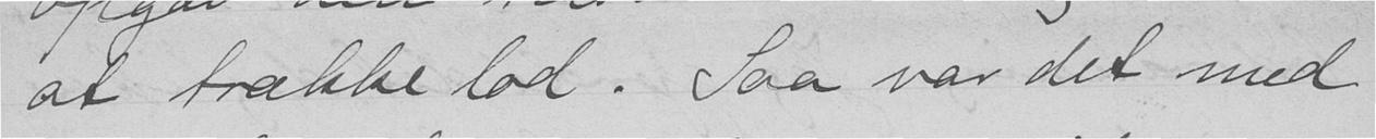at trække lod. Saa var det med
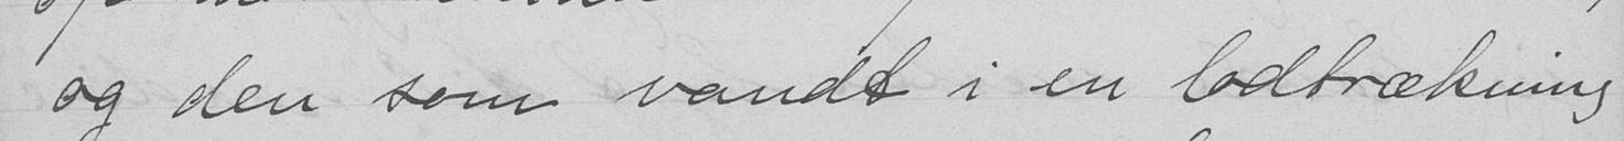og den som vandt i en lodtrækning
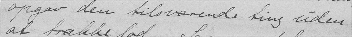opgav den tilsvarende ting uden
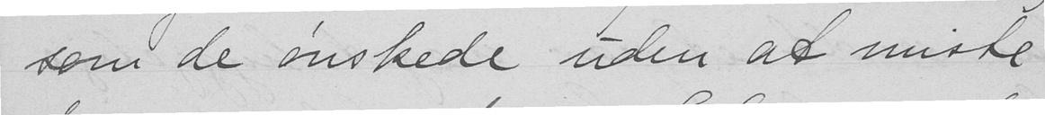som de ønskede uden at miste
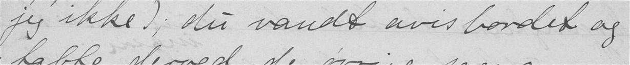jeg ikke); du vandt avisbordet og
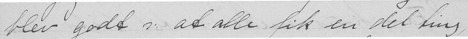blev godt, at alle fik en del ting,
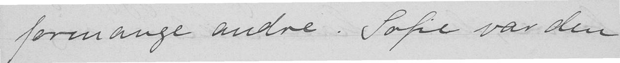formange andre. Sofie var den
